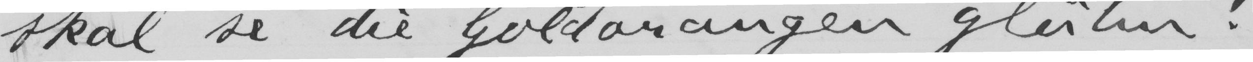skal se die Goldorangen glühn!
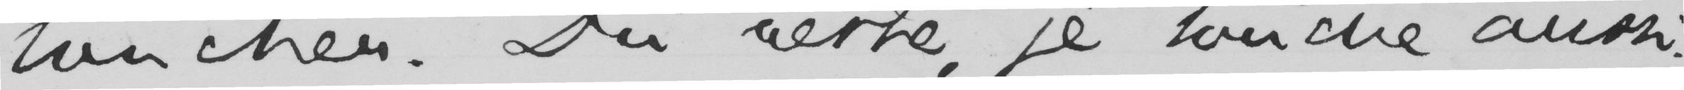toucher. Du reste, je touche aussi,
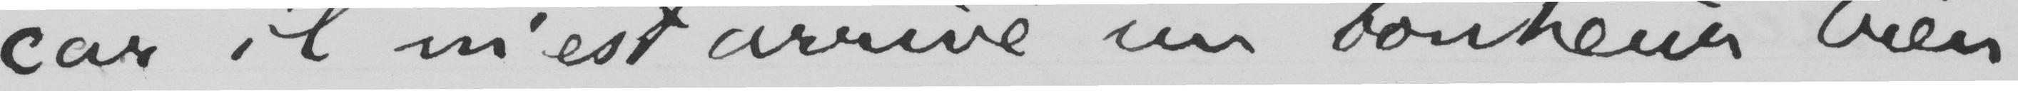car il m’est arrivé un bonheur bien
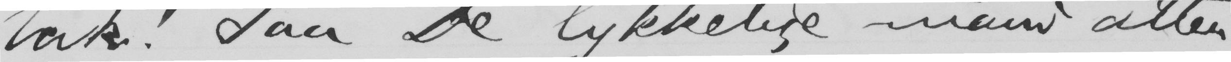tak! Saa De lykkelige mand atter
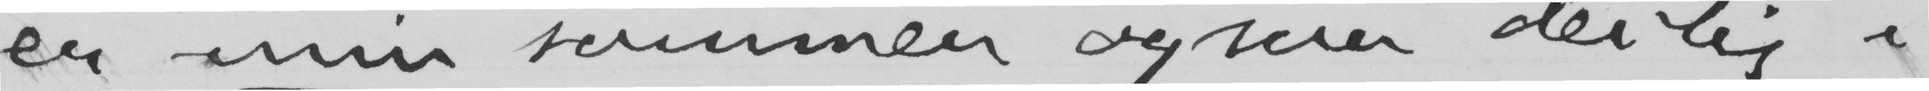er min sommer ogsaa deilig i
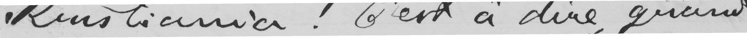Kristiania! C’est à dire, quand
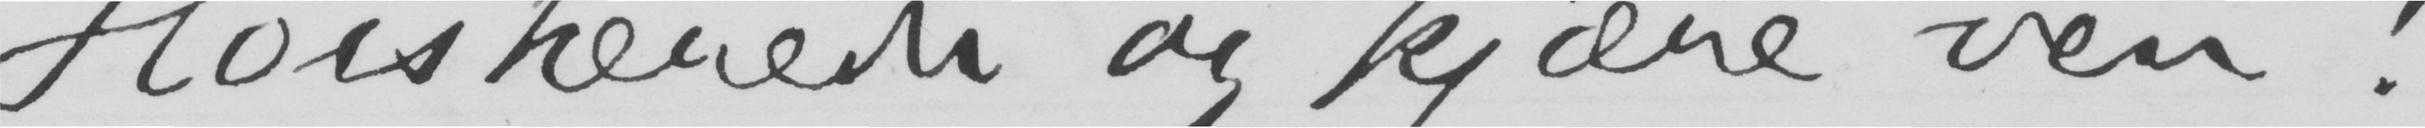Høistærede og kjære ven!
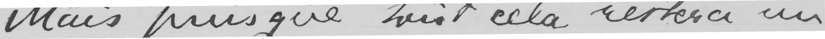Mais puisque tout cela restera un
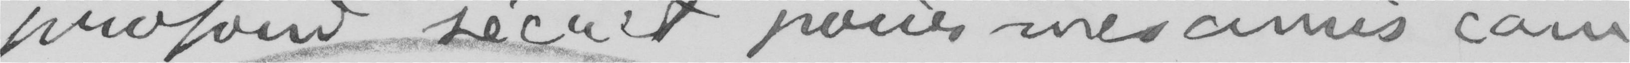profond sécret pour mes amis com-
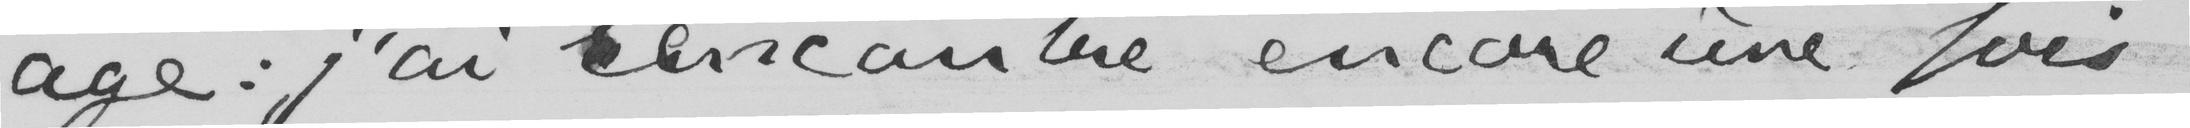age: j’ai rencontré encore une fois
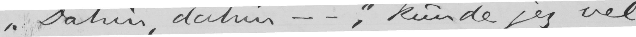«Dahin, dahin…» kunde jeg vel
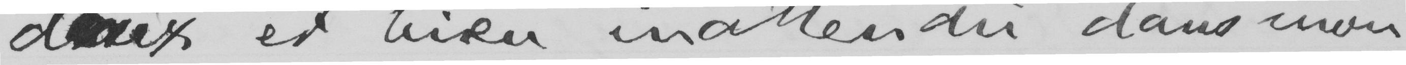doux et bien inattendu dans mon
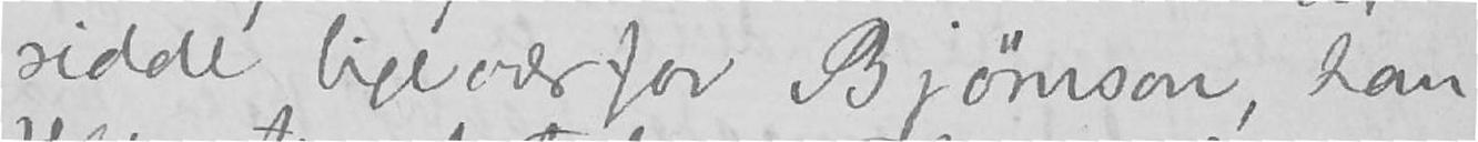sidde ligeoverfor Bjørnson, han
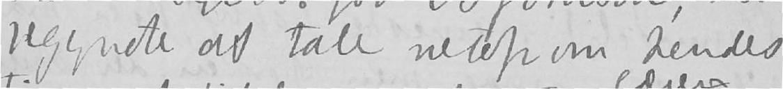begyndte at tale netop om hendes
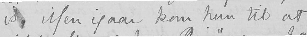os. Men igaar kom hun til at
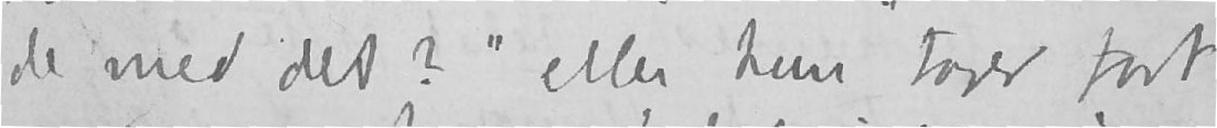de med det?» eller hun tager fort
